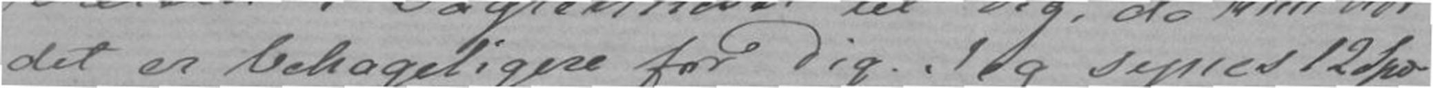det er behagelig for Dig. Jeg synes 12 Spd
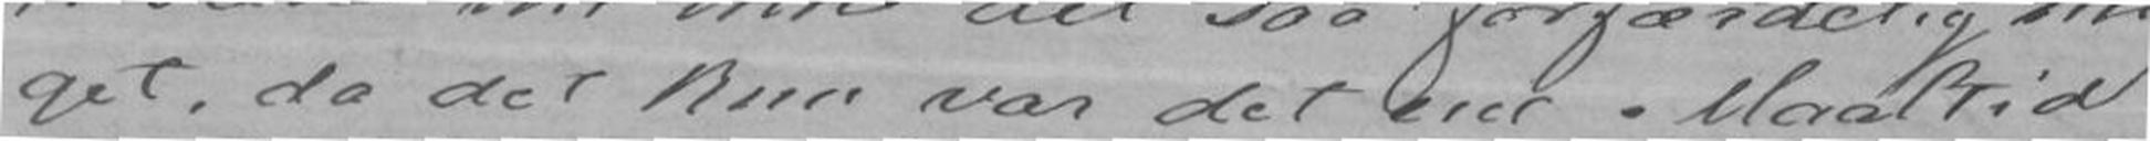get, da det kun var det ene Maaltid
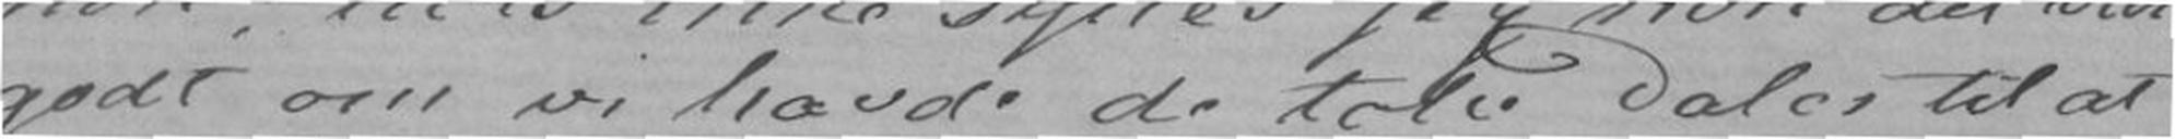godt om vi havde de tolv Deler til at
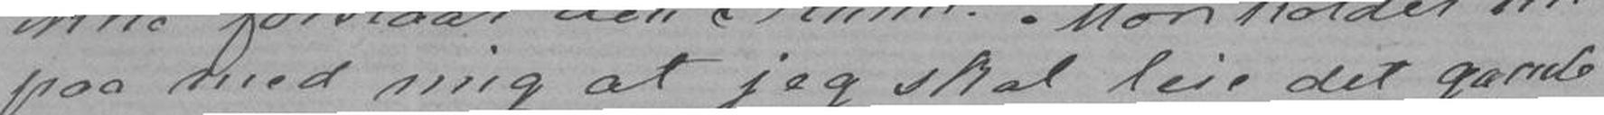paa med mig at jeg skal leie det gamle
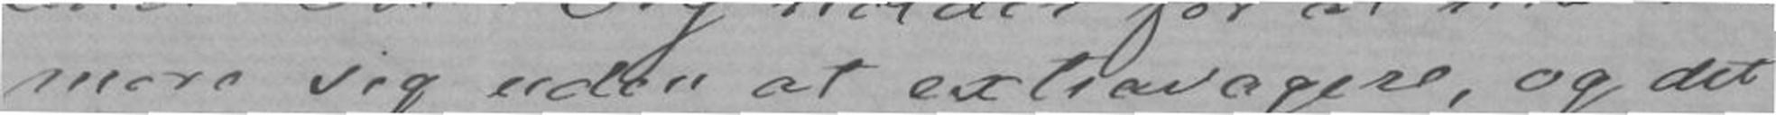more sig uden at extrasagere, og det
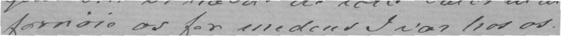fornøie os for medens I var hos os.
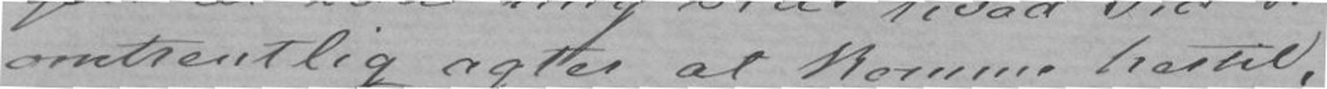omtrentlig agtes at komme hertil,
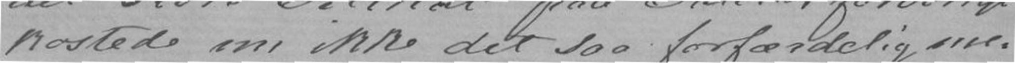kostede nu ikke det saa forfærdelig me-
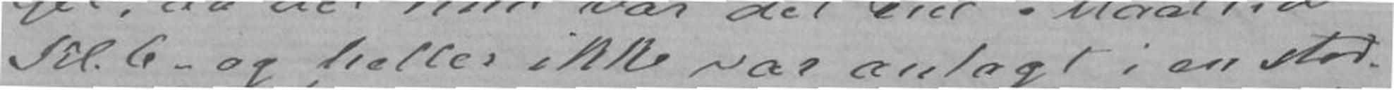Kl.6 - og heller ikke var anlagt i en stod-
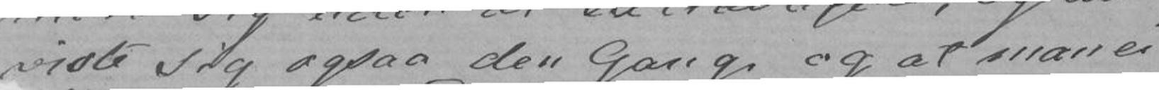viste sig ogsaa den Gang og at man ei
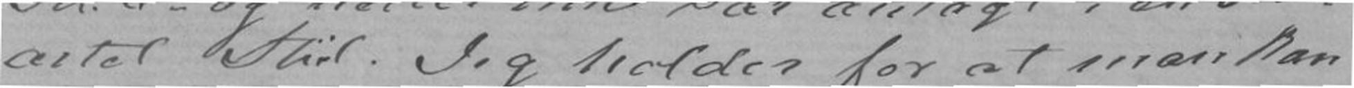artet Stil. Jeg holder for at man kan
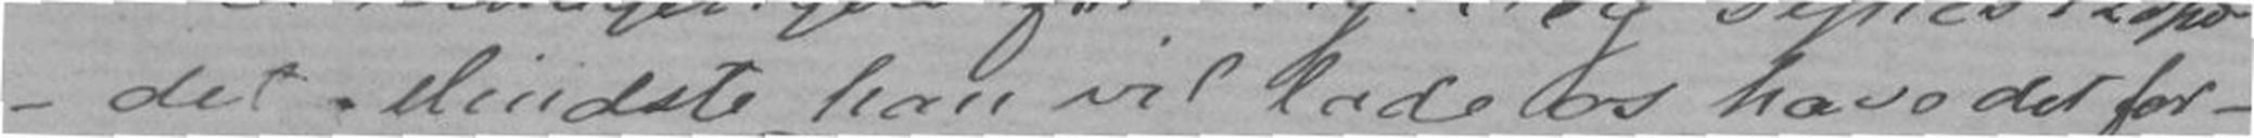- det Mindste han vil lade os have det for -
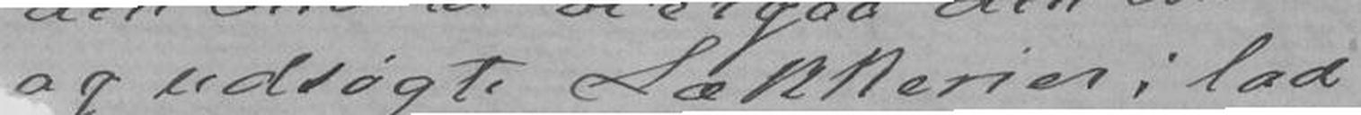og udsøgte Lækkerier i lad
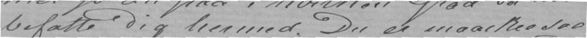befatte Dig hermed. Du er maskee saa
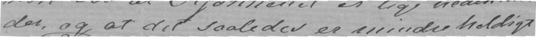der, og at det saaledes er mindre heldigt
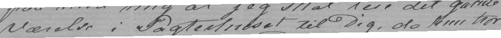Værelse i Pagterhuset til Dig, da hun tror
